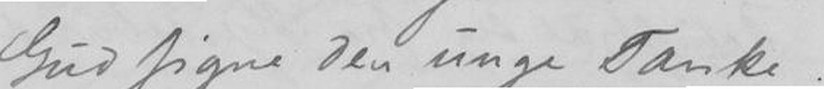Gud signe den unge Tanke!
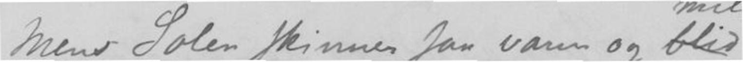Mens Solen skinner saa varm og mild blid
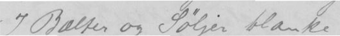I Bælter og Søljer blanke,
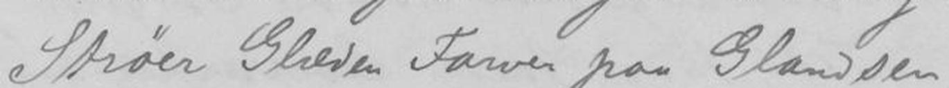Strøer Glæden Farver paa Glandsen,
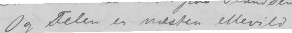Og Felen er næsten ellevild;
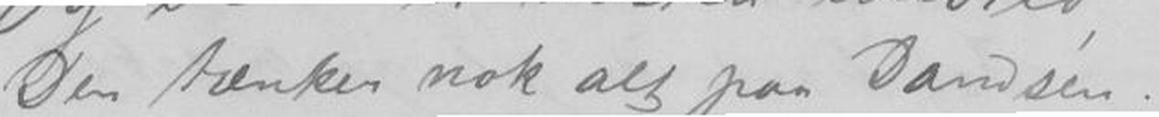Den tænker nok alt paa Dandsen;
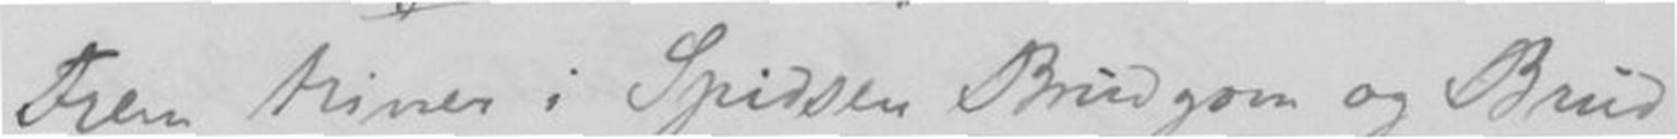Frem triver i Spidsen Brudgom og Brud
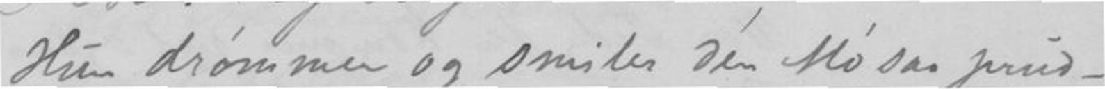Hun drømmer og smiler den Mø saa prud -
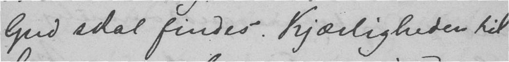Gud skal findes. Kjærligheden til
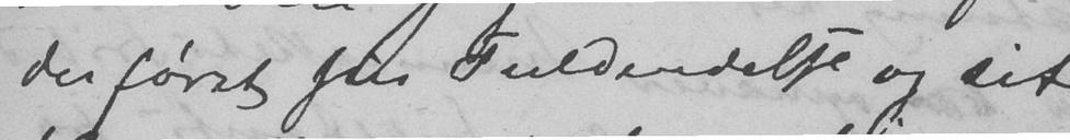der først fra Fuldendelse og sit
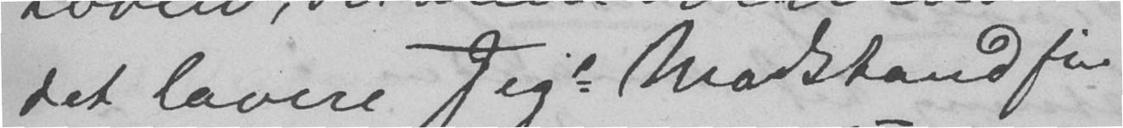det lavere Jeg = Modstand fin-
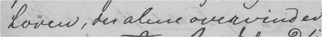Loven, der alene overvinder
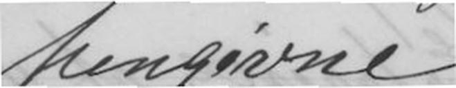hengivne
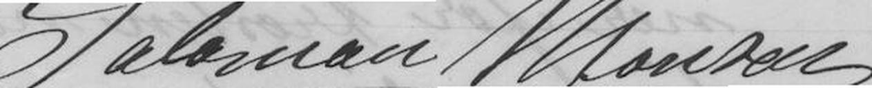Salomon Monsen
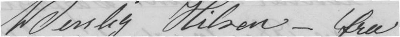Venlig Hilsen - fra
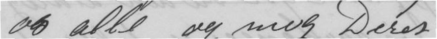os alle og mig Deres
